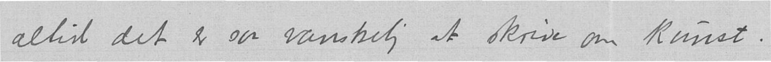altid det er saa vanskelig at skrive om kunst.
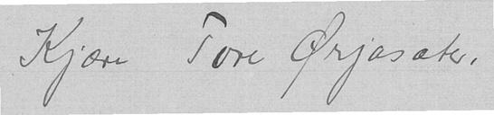Kjære Tore Ørjasæter,
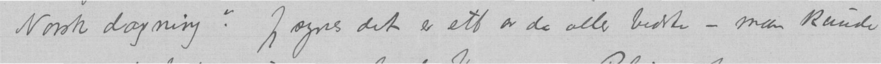Norsk dagning? Jeg synes det er ett av de aller bedste – man kunde
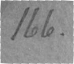166.
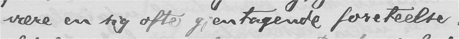være en sig ofte gjentagende foreteelse.
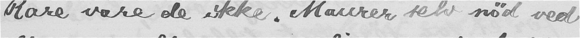Rare vare de ikke. Maurer selv nød ved
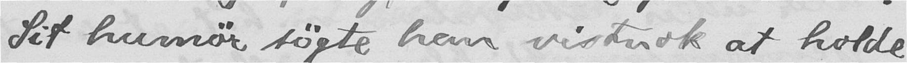Sit humør søgte han vistnok at holde
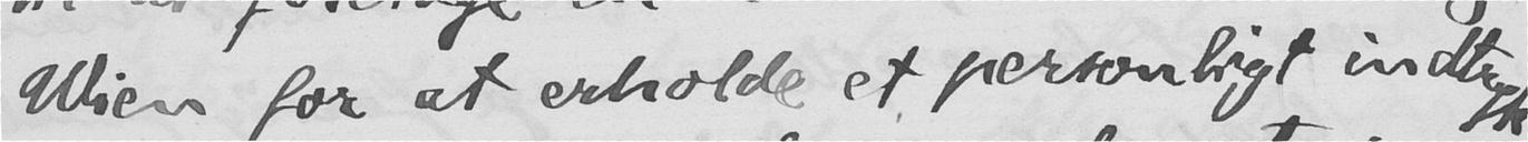Wien for at erholde et personligt indtryk
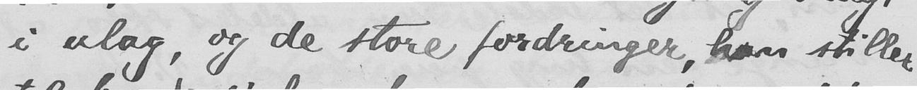i ulag, og de store fordringer, han stiller
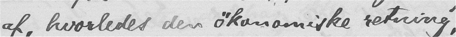af, hvorledes den økonomiske retning,
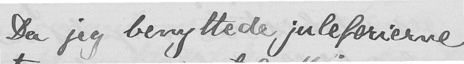Da jeg benyttede juleferierne
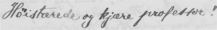Høistærede og kjære professor!
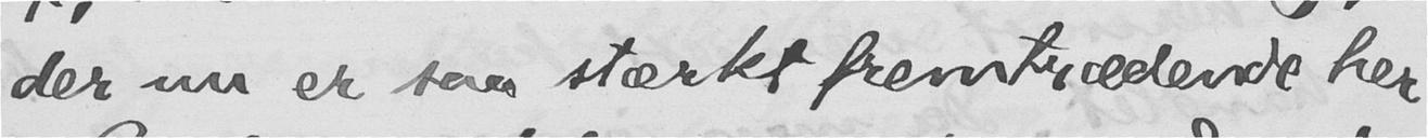der nu er saa stærkt fremtrædende her
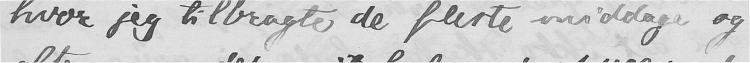hvor jeg tilbragte de fleste middage og
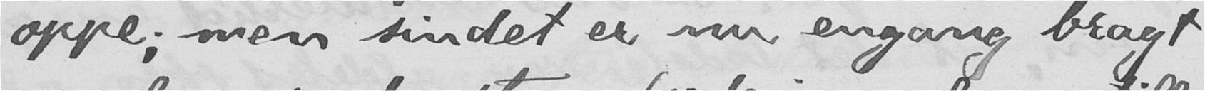oppe; men sindet er nu engang bragt
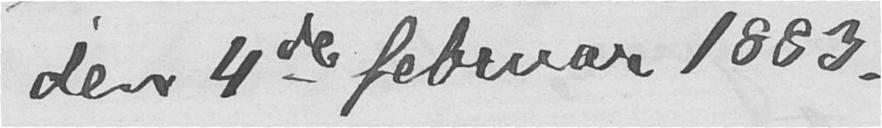den 4de februar 1883.
